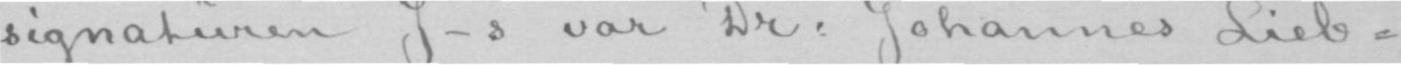signaturen J-s var Dr: Johannes Lieb-
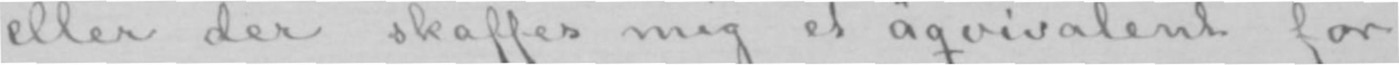eller der skaffes mig et äqvivalent for
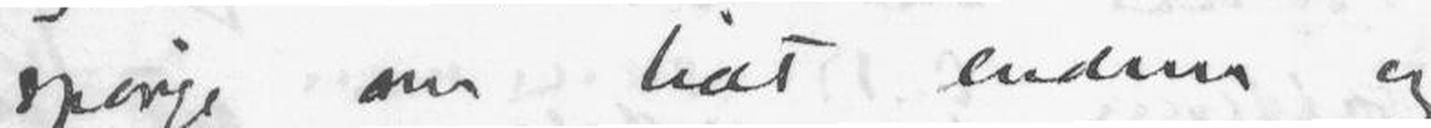spørge om lidt endnu og
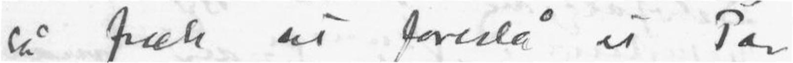så fræk at foreslå et Par
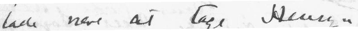lade være at tage Hensyn
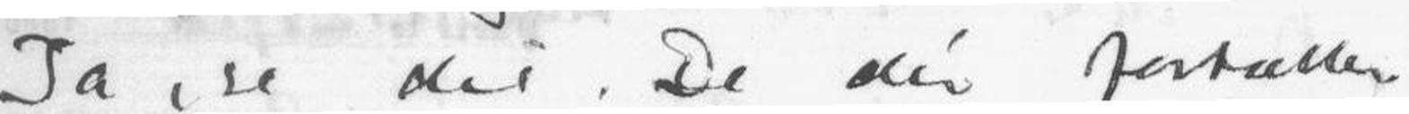Ja, se det. De dér fortæller
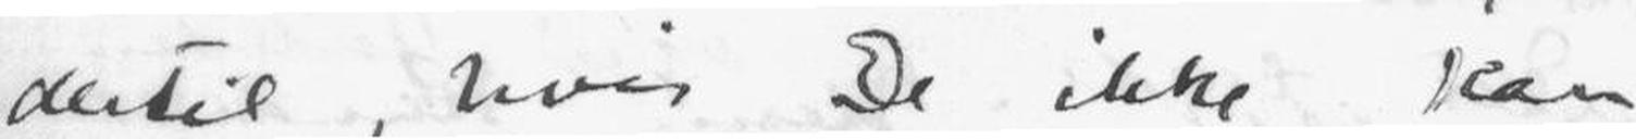dertil, hvis De ikke kan
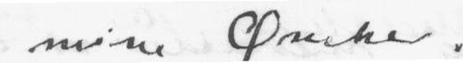mine Ønsker.
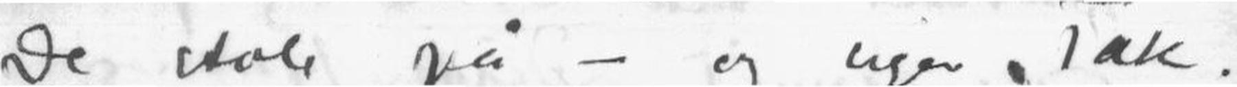De stole på - og siger Tak.
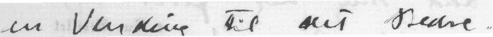en Vending til det Bedre.
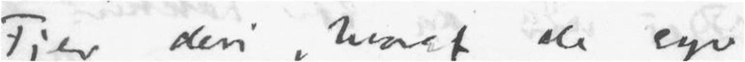Fjer deri, hvoraf de syv
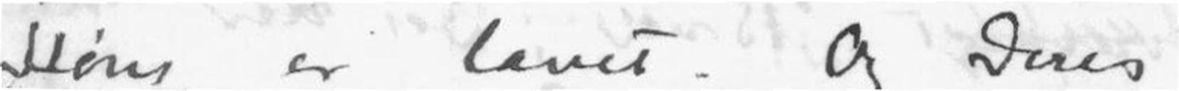Høns er lavet. Og Deres
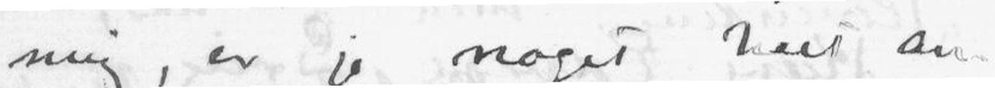mig, er jo noget helt an-
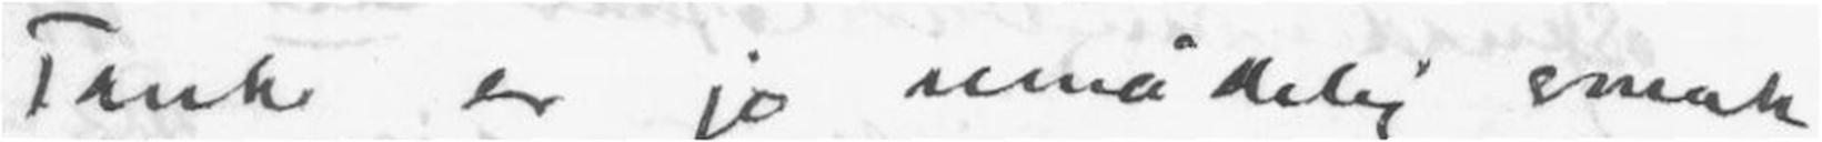Tanke er jo umådelig smak
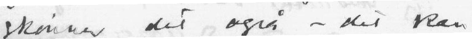skønner det også - det kan
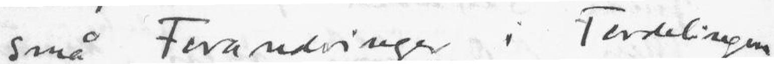små Forandringer i Fordelingen
